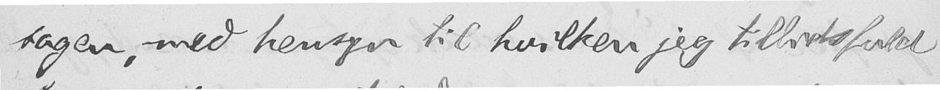sagen, med hensyn til hvilken jeg tillidsfuld
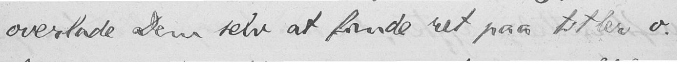overlade Dem selv at finde ret paa titler o.
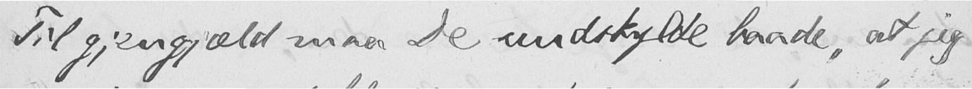Til gjengjæld maa De undskylde baade, at jeg
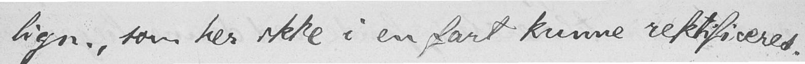lign., som her ikke i en fart kunne rektificeres.
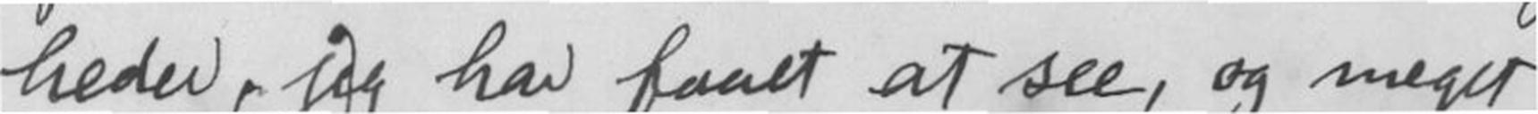heder, jeg har faaet at see, og meget
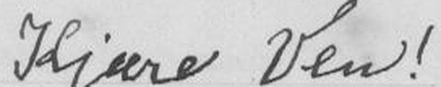Kjære Ven!
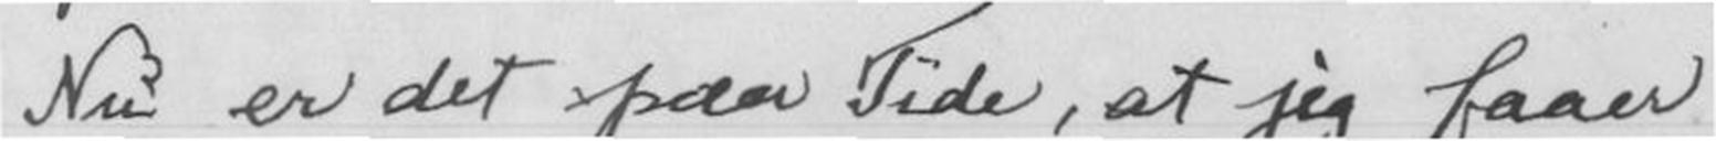Nu er det paa Tide, at jeg faaer
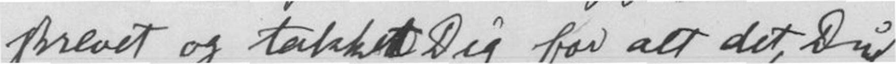skrevet og takket Dig for alt det, Du
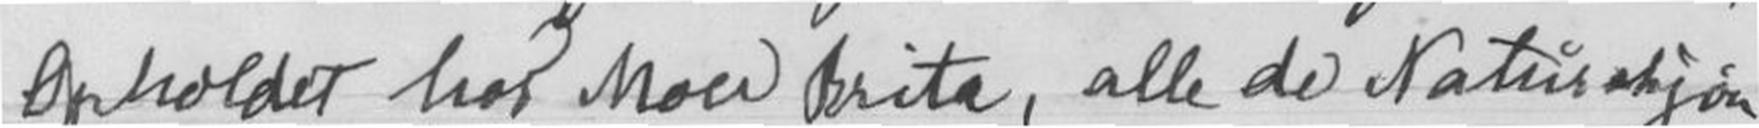Opholdet hos Moer Brita, alle de Naturskjøn-
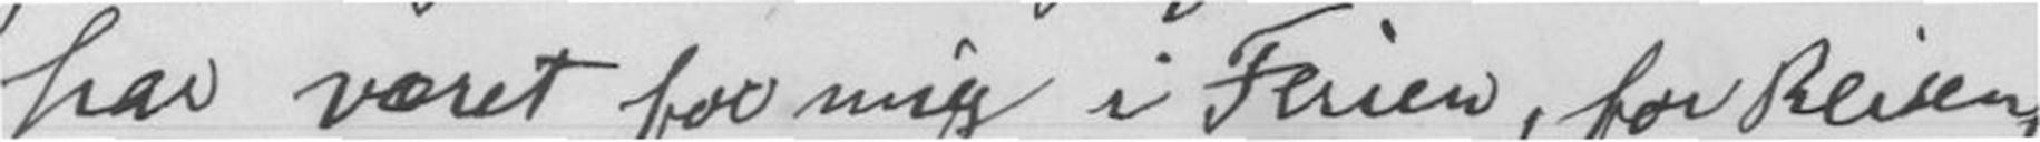har været for mig i Ferien, for Reisen,
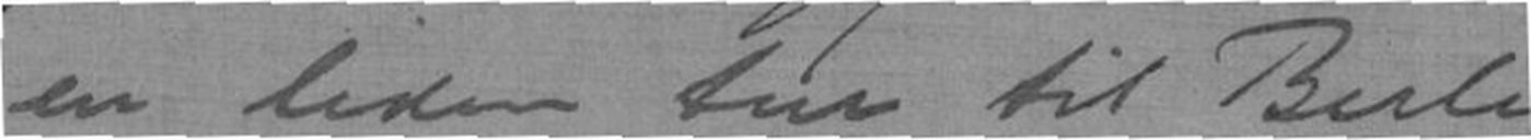en liden tur til Berlin
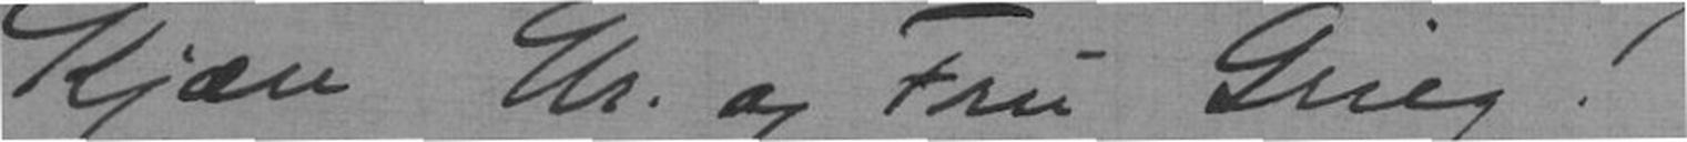Kjære Hr. og Fru Grieg!
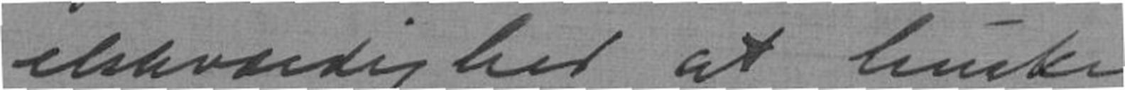elskværdighed at huske
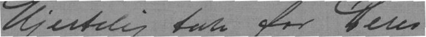Hjertelig tak for Deres
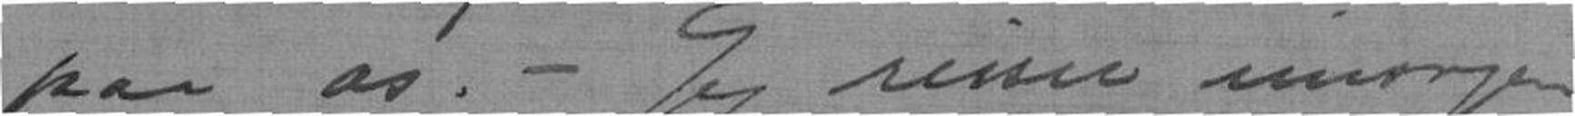paa os. - Jeg reiser imorgen
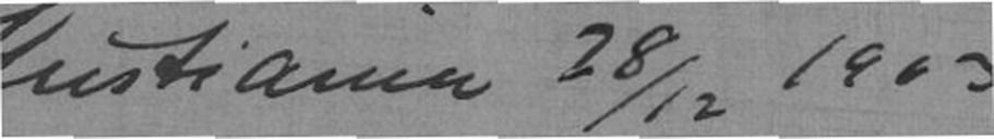Kristiania 28/12 1903
